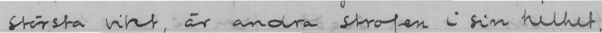största vikt, är andra strofen i sin helhet,
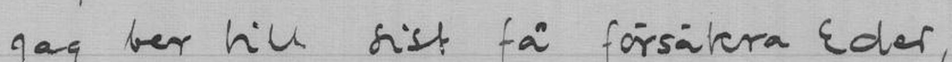Jag ber till sist få försäkra Eder,
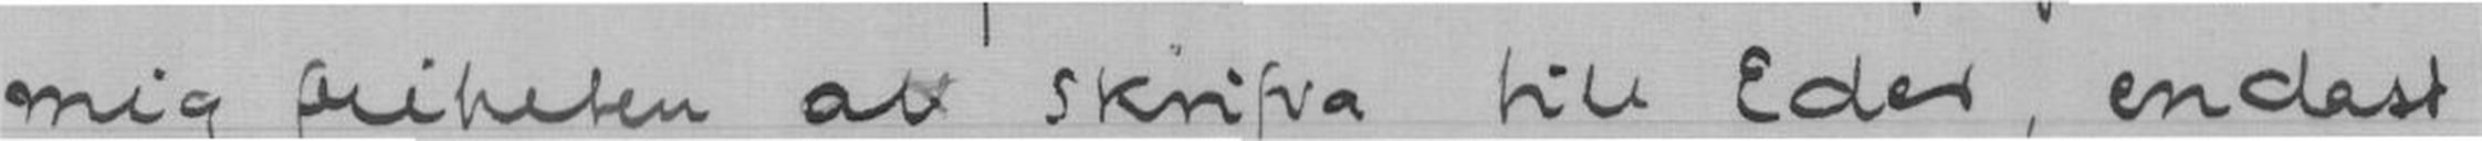mig friheten att skrifva till Eder, endast
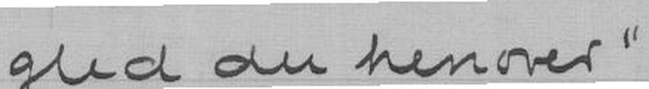gled du henover" - -
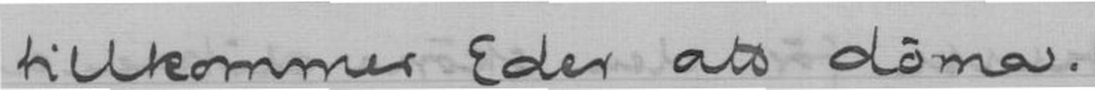tillkommer Eder att döma.
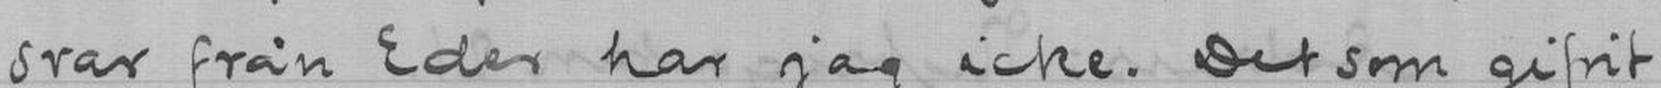svar från Eder har jag icke. Det som gifvit
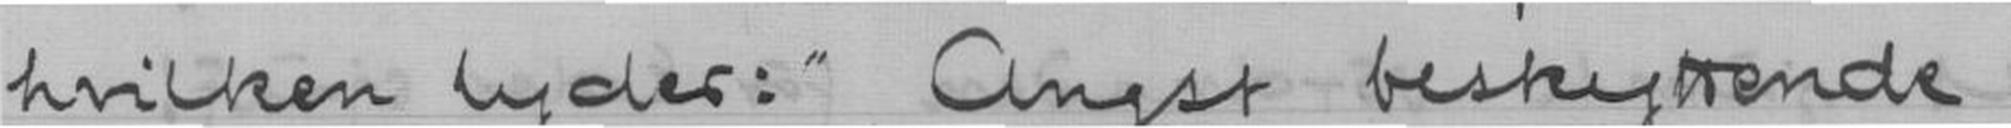hvilken lyder: " Angst beskyttende
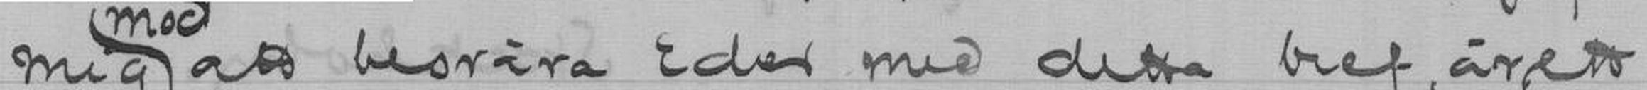mig att besvära Eder med detta bref, är ett
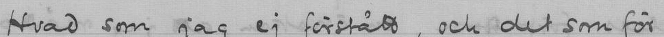Hvad som jag ej förstått, och det som för
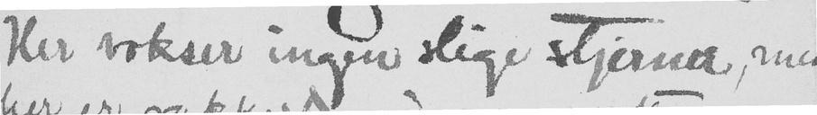Her vokser ingen slige stjerner, men
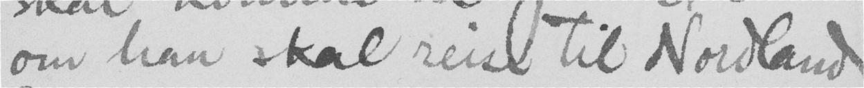om han skal reise til Nordland
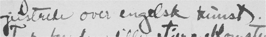geistrede over engelsk kunst.
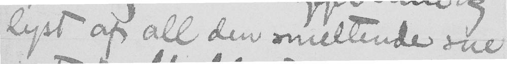lyst af all den smeltende sne
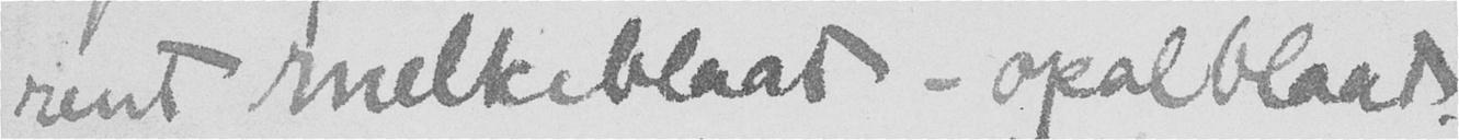rent melkeblaat - opalblaat.
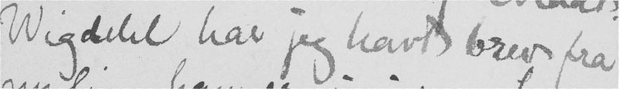Wigdehl har jeg havt brev fra
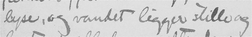lyse, og vandet ligger stille og
